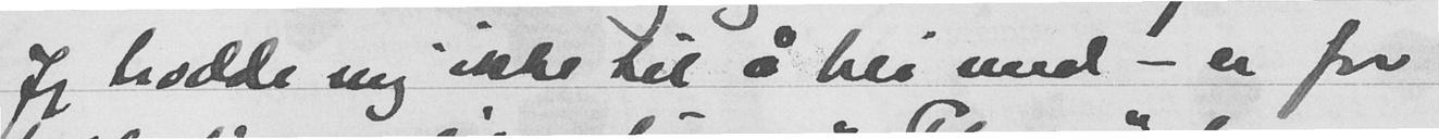Jeg trodde mig ikke til å bli med - er for
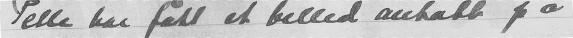Pelle har fått et billed antatt på
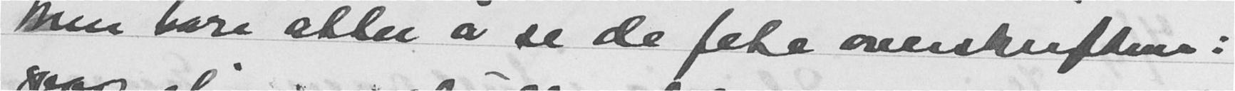Men bare atter å se de fete overskriftene:
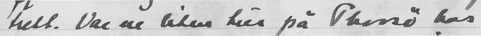trett. Var en liten tur på Thorsø hos
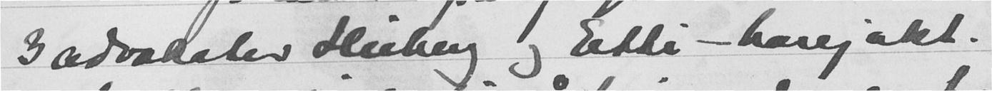3 advokater Heiberg og Elli - harejakt.
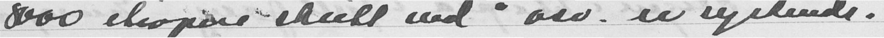"8000 etiopere skutt ved" osv. er rystende.
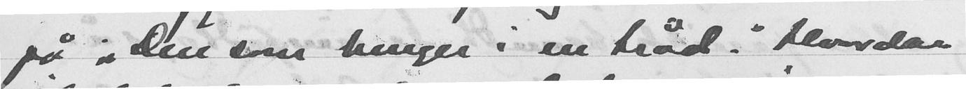på "Den som henger i en tråd." Hvordan
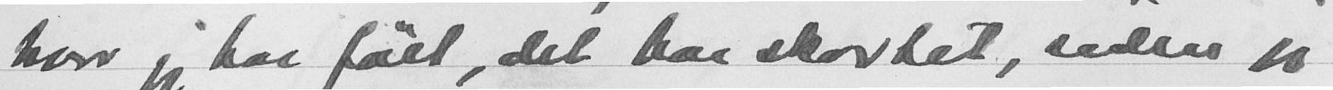hvor jeg har falt, det har skortet, siden jeg
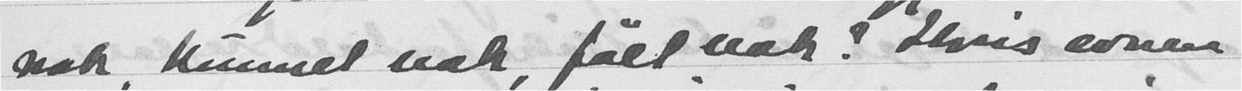nok, kunnet nok, følt nok? Hvis evnen
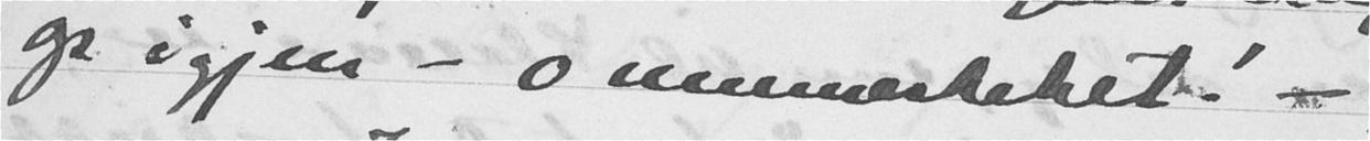op igjen - O menneskehet! -
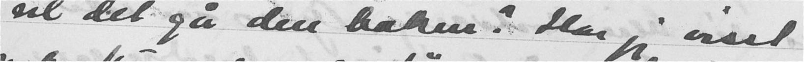vil det gå den boken? Har jeg visst
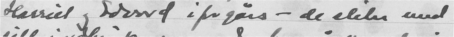Harriet og Edvard i forgårs - de sliter med
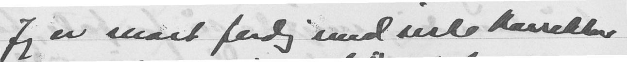Jeg er snart ferdig med siste korrektur
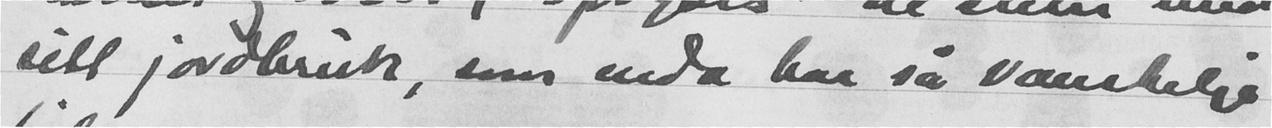sitt jordbruk, som enda har så vanskelige
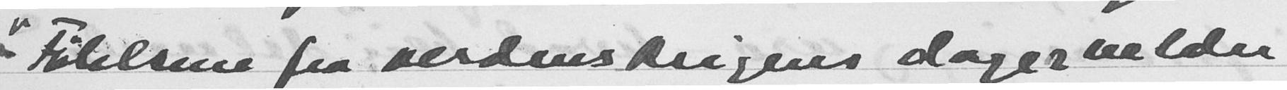Følelsene fra verdenskrigens dager velder
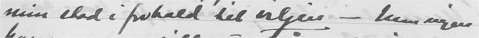min stod i forhold til viljen - Men ingen
